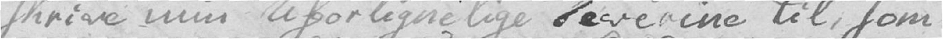skrive min Uforlignelige Severine til, som
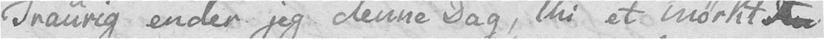Traurig ender jeg denne Dag, thi et mørkt
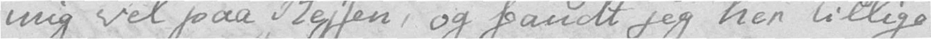mig vel paa Rejsen, og fandt jeg her tillige
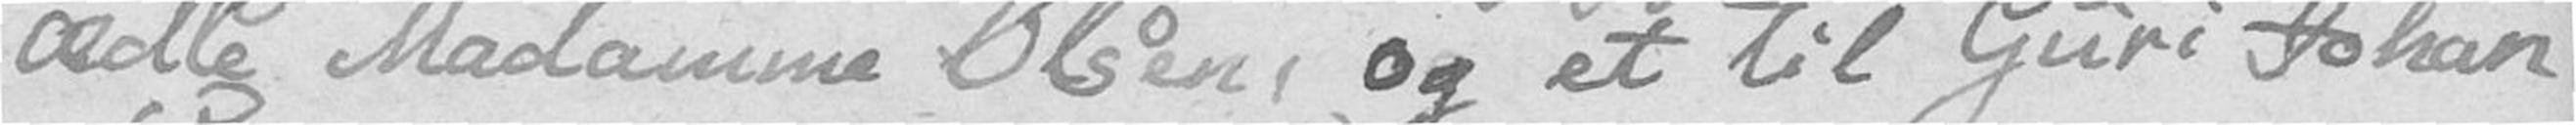ædle Madamme Olsen, og et til Guri Johan
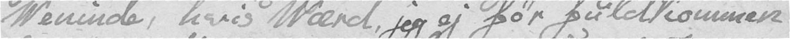Veninde, hvis Værd, jeg ej før fuldkommen
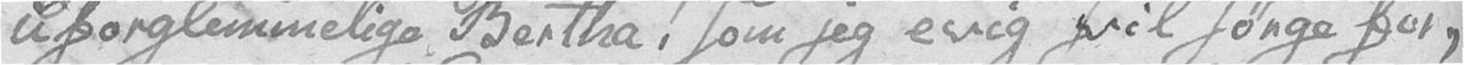uforglemmelige Bertha! som jeg evig vil sørge for,
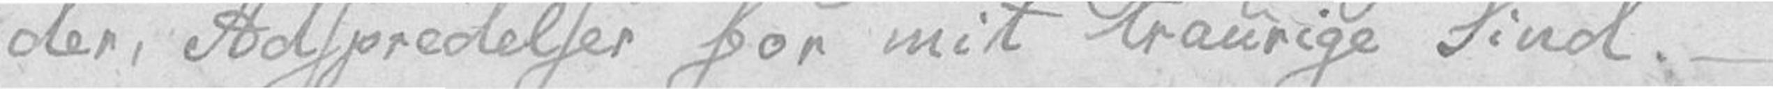der, Adspredelser for mit traurige Sind. –
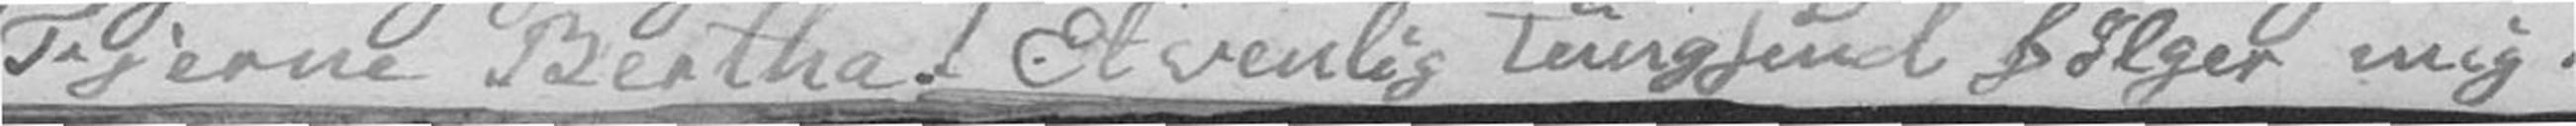Fjerne Bertha! Et venlig Tungsind følger mig.
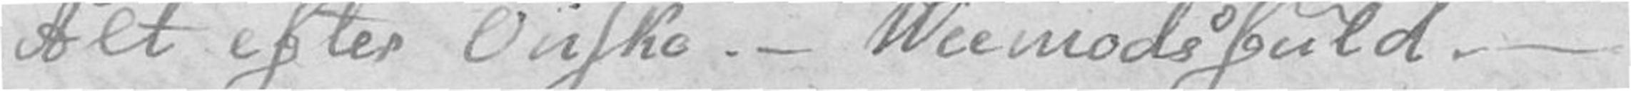Alt efter Ønske. – Veemodsfuld. –
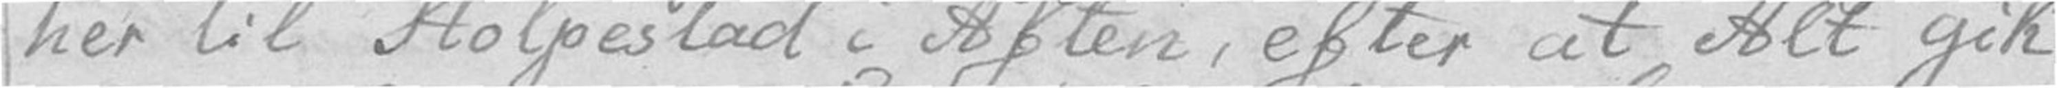her til Stolpestad i Aften, efter at Alt gik
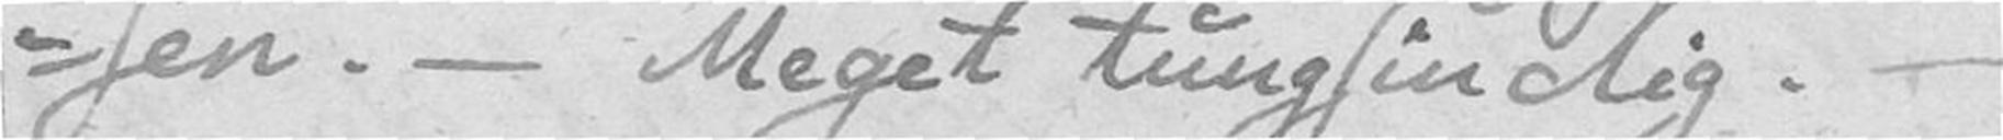-sen. – Meget tungsindig. –
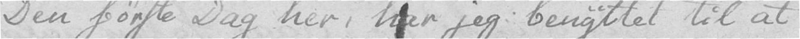Den første Dag her, har jeg benyttet til at
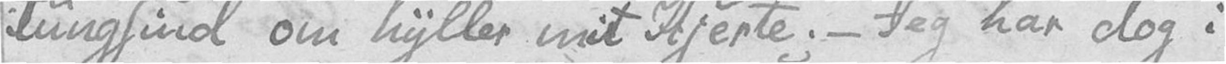tungsind om hyller mit Hjerte. – Jeg har dog i
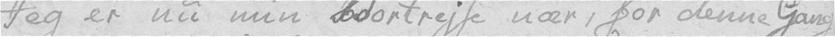Jeg er nu min Bortrejse nær, for denne Gang
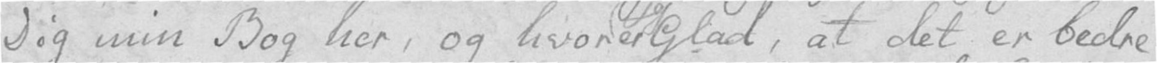Dig min Bog her, og hvor er jeg Glad, at det er bedre
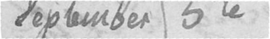September 5te
